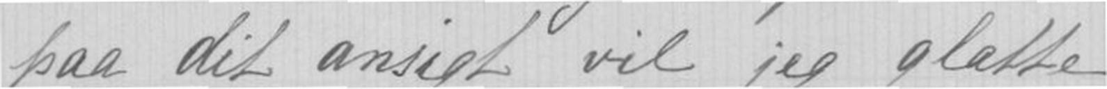paa dit ansigt vil jeg glatte,-
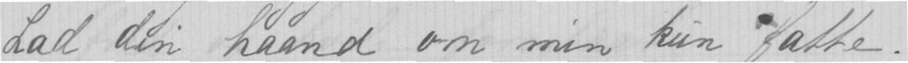Lad din haand om min kun fatte.
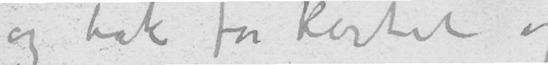og tak for kortet og
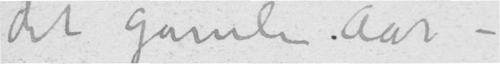det gamle Aar –
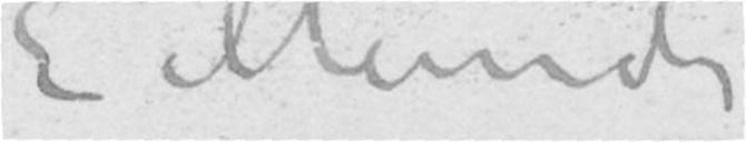E Munch
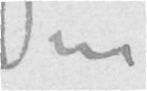Din
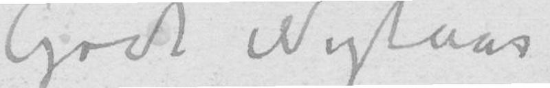Godt Nytaar
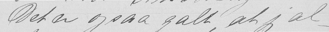Det er ogsaa galt at jeg al-
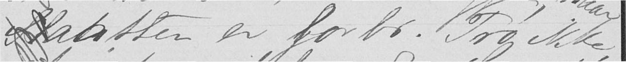Slaatten er forbi. Tro ikke
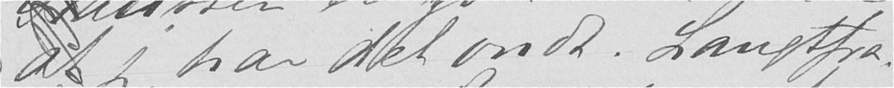at jeg har det ondt. Langtfra.
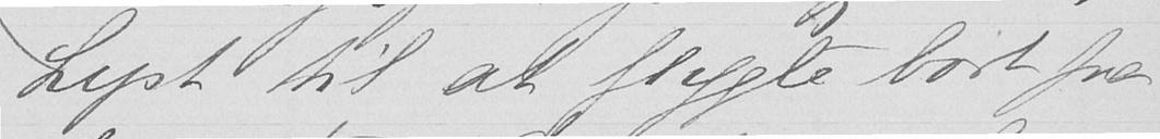Lyst til at flygte bort fra
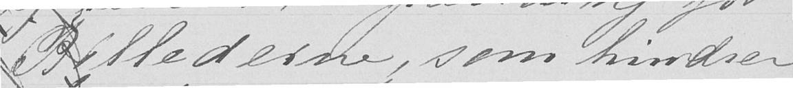Billederne, som hindrer
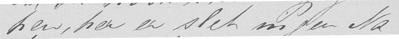hen, her er slet ingen Na-
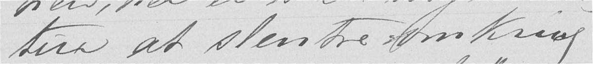tur at slentre omkring
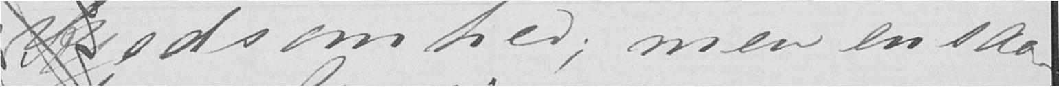Kedsomhed; men en saa-
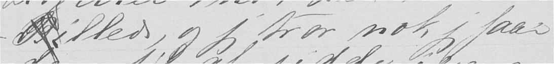Billede, og jeg tror nok jeg faar
